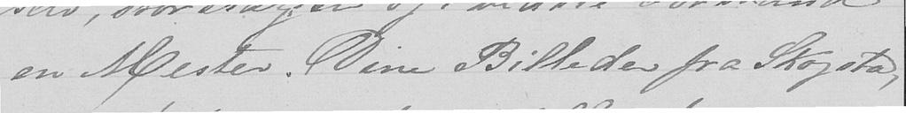en Mester. Dine Billeder fra Skogstad,
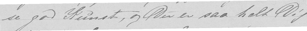se god Kunst, og Du er saa helt Dig
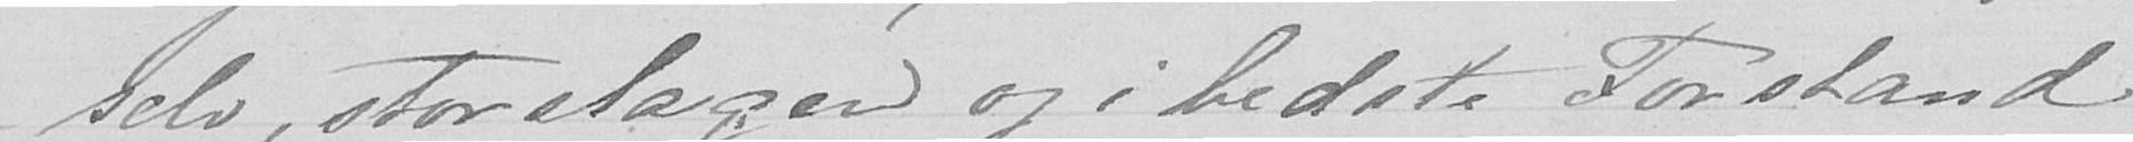selv, storslagen og i bedste Forstand
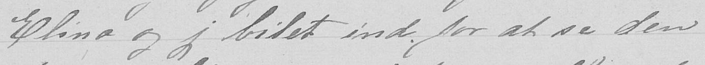Elina og jeg bilet ind, for at se den
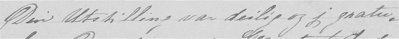Din Utstilling var deilig og jeg gratu-
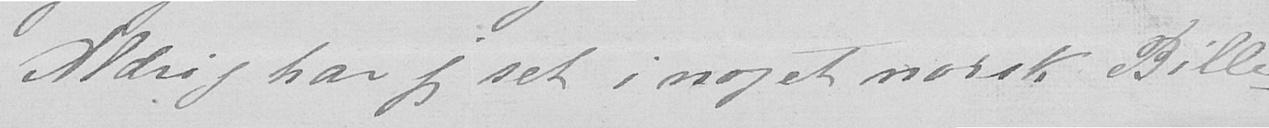Aldrig har jeg set i noget norsk Bille-
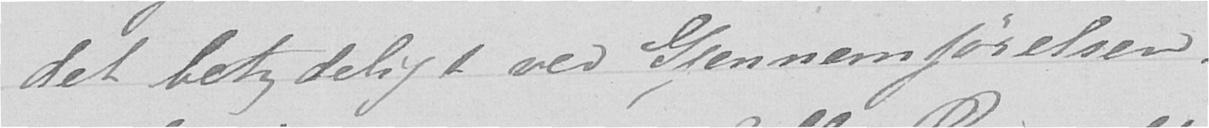det betydeligt ved Gjennemførelsen.
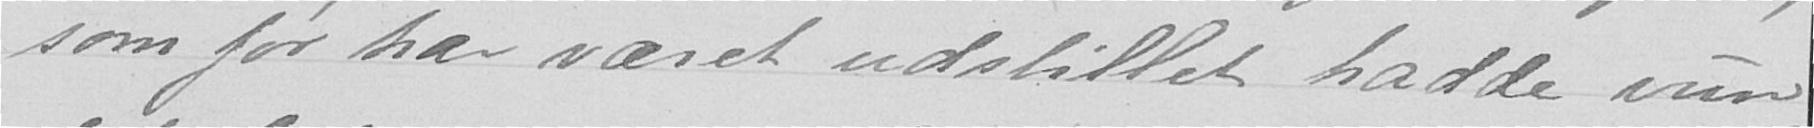som før har været udstillet hadde vun-
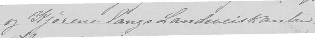og Kjørene langs Landeveiskanten.
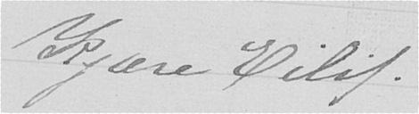Kjære Eilif
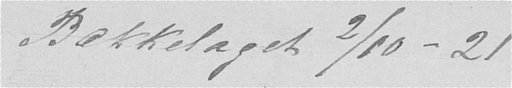Bækkelaget 5/10- 21
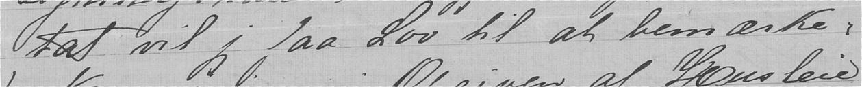tat vil jeg faa Lov til at bemærke,
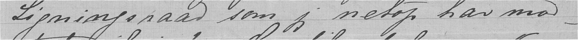Ligningsraad som jeg netop har mod-
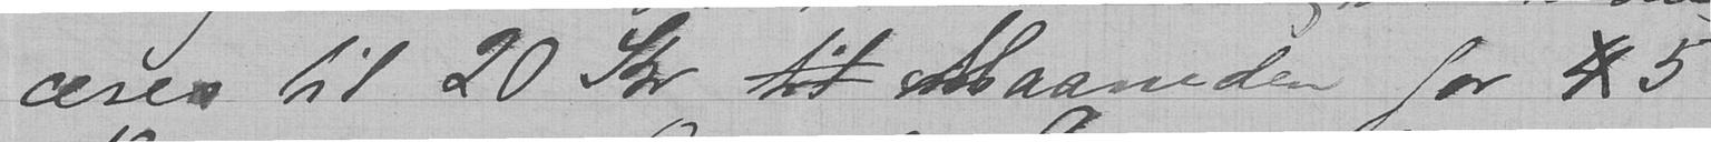ceres til 20 Kr til Maaneden for 45
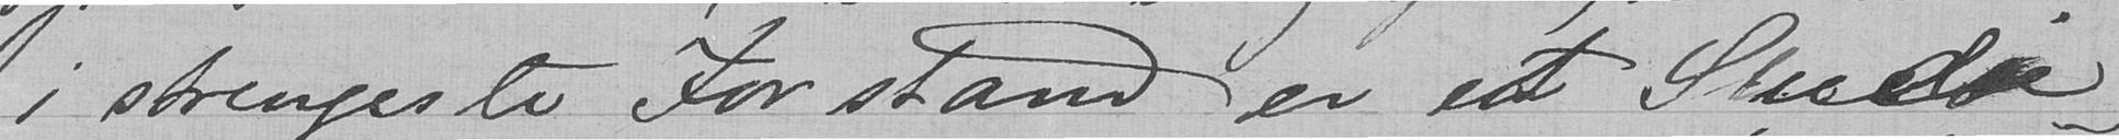i strengeste Forstand er et Studie-
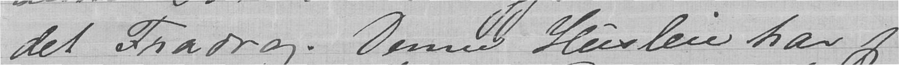det Fradrag. Denne Husleie har jeg
